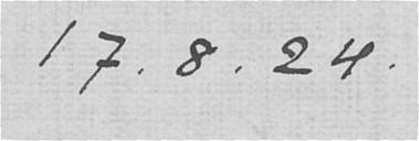17.8.24.
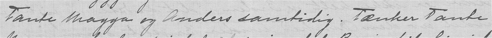tante Magga og Anders samtidig. Tænker Tante
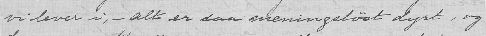vi lever i, - alt er saa meningsløst dyrt, og
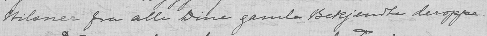Hilsner fra alle Dine gamle Bekjendte deroppe.
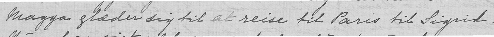Magga glæder sig til at reise til Paris til Sigrid.
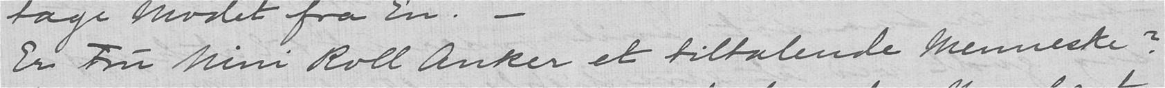Er Fru Nini Roll Anker et tiltalende Menneske?
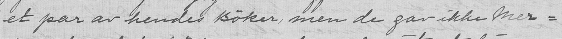et par av hendes Bøker, men de gav ikke mer-
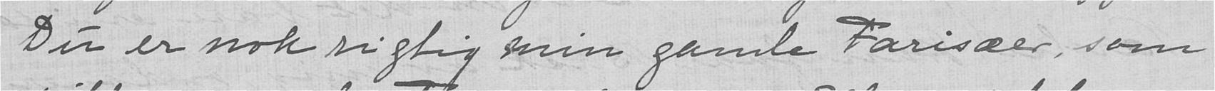Du er nok rigtig min gamle Farisæer, som
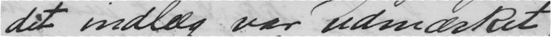dit indlæg var udmærket,
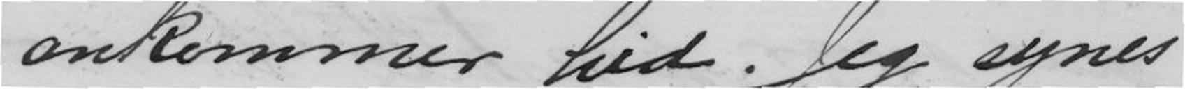ankommer hid. Jeg synes
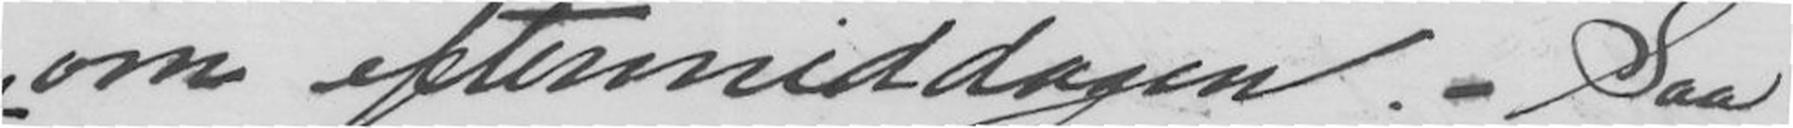om eftermiddagen. Saa
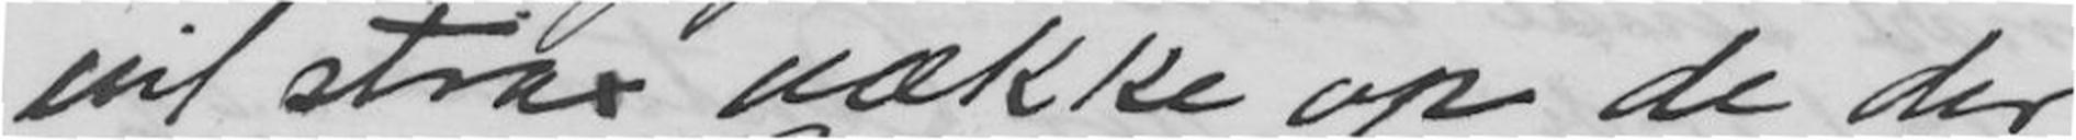vil strax vække op de der
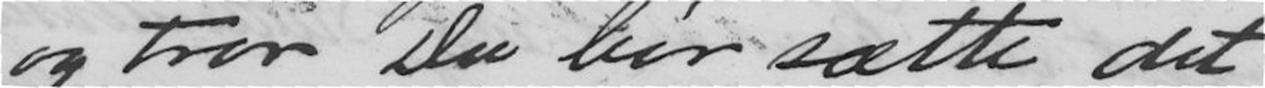og tror Du bør sætte det
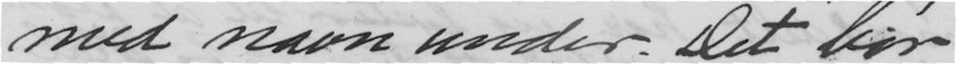med navn under. Det bør
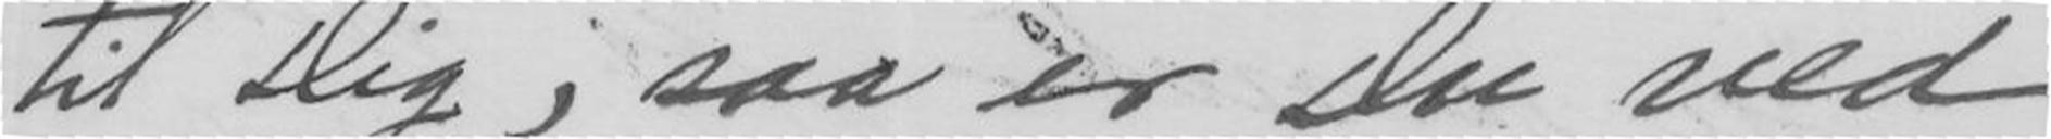til Dig, saa er Du ved
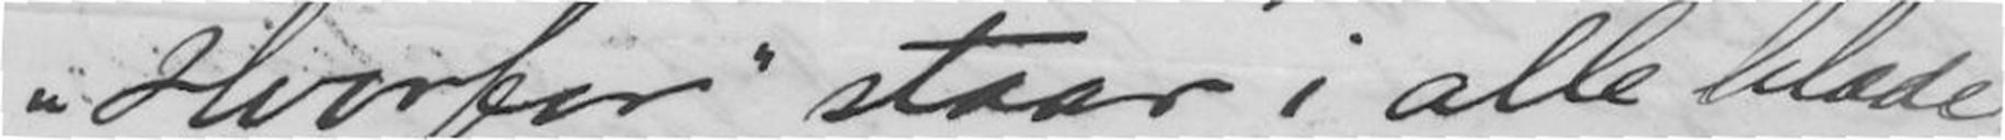"Hvorfor" staar i alle blade
